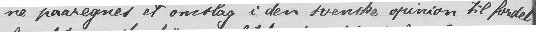ne paaregnes et omslag i den svenske opinion til fordel
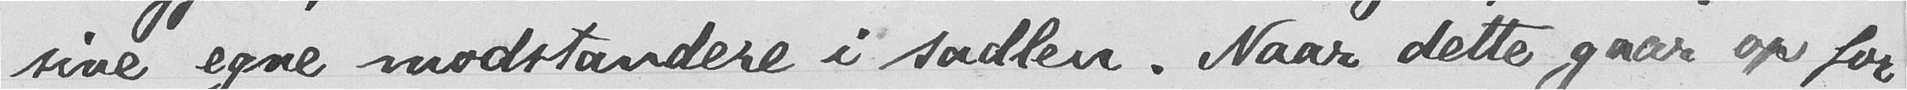sine egne modstandere i sadlen. Naar dette gaar op for
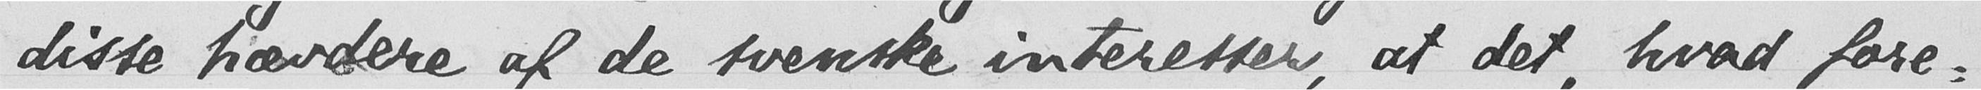disse hævdere af de svenske interesser, at det, hvad fore-
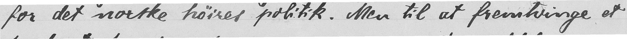for det norske høires politik. Men til at fremtvinge et
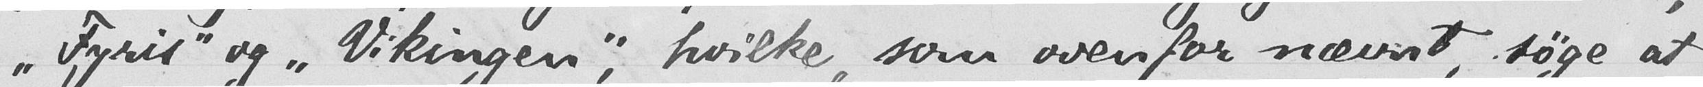"Fyris" og "Vikingen", hvilke, som ovenfor nævnt, søge at
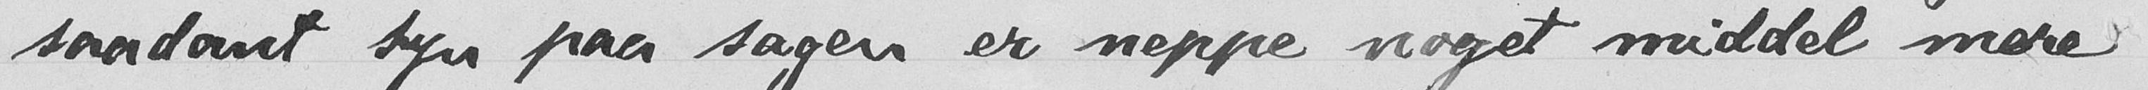saadant syn paa sagen er neppe noget middel mere
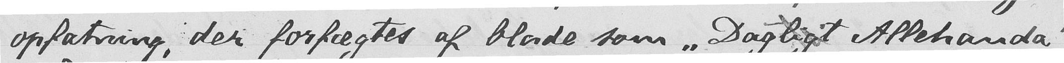opfatning, der forfægtes af blade som "Dagligt Allehanda",
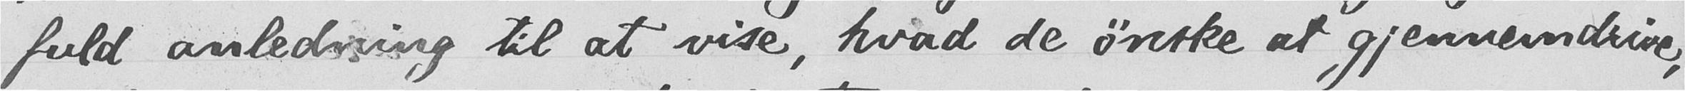fuld anledning til at vise, hvad de ønske at gjennemdrive,
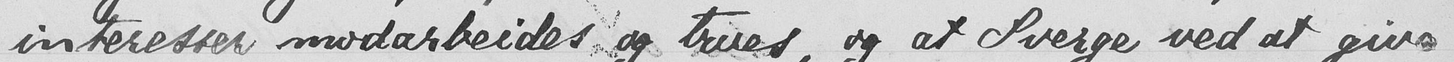interesser modarbeides og trues, og at Sverge ved at give
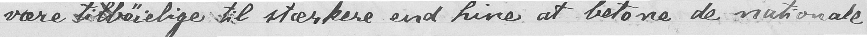være tilbøielige til stærkere end hine at betone de nationale
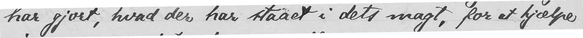har gjort, hvad der har staaet i dets magt, for at hjælpe
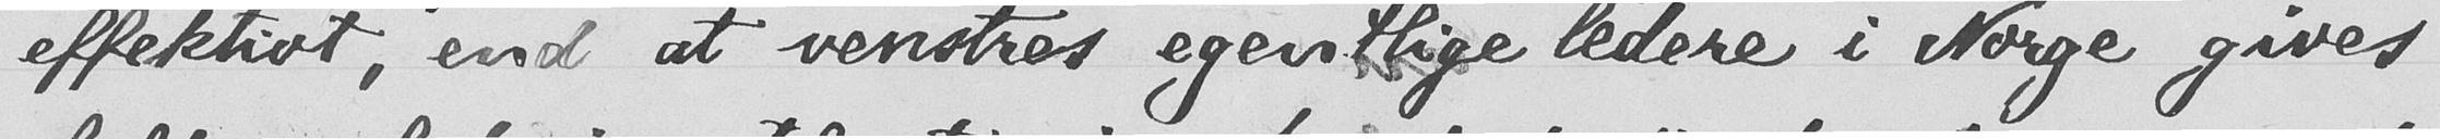effektivt, end at venstres egentlige ledere i Norge gives
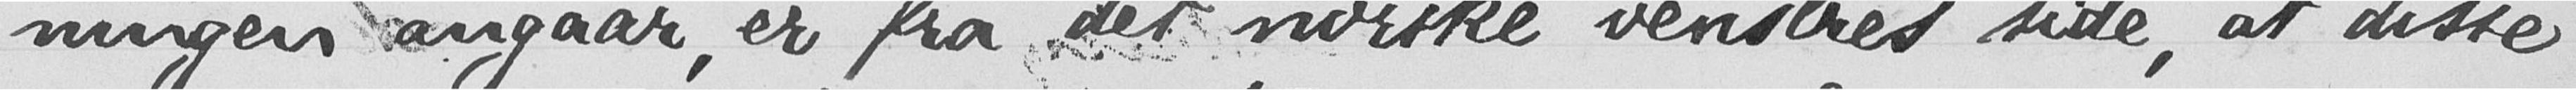ningen angaar, er fra det norske venstres side, at disse
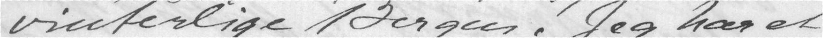vinterlige Bergen! Jeg har et
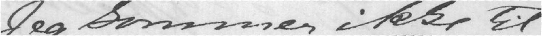Jeg kommer ikke til
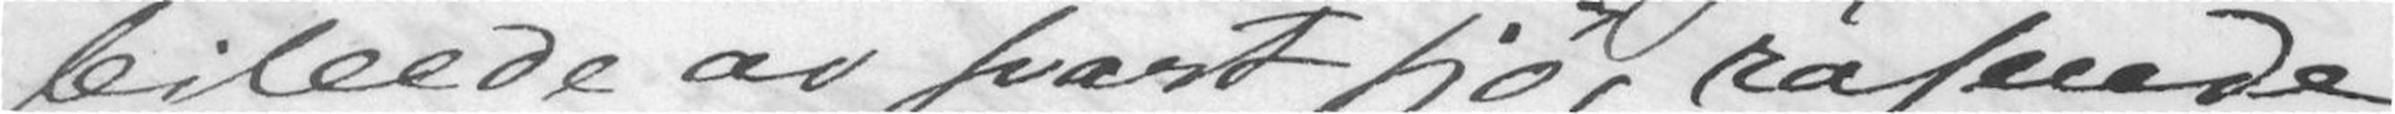billede av svart sjø, rasende
